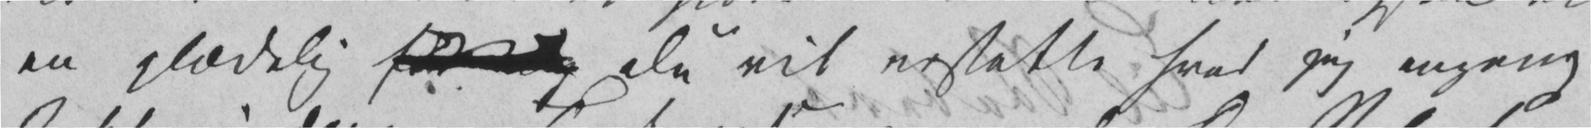en glædelig <gap/> Du vil erstatte hvad jeg engang
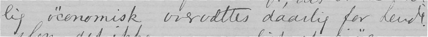lig øconomisk overvættes daarlig for hende.
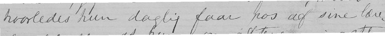hvorledes hun daglig faar ros af sine lære-
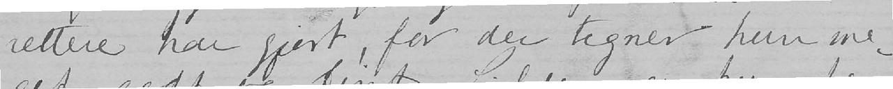rettere har gjort, for der tegner hun me-
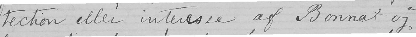tection eller interesse af Bonnat og
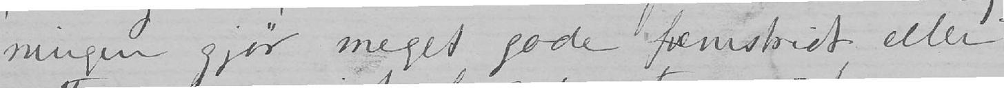ningen gjør meget gode fremskridt eller
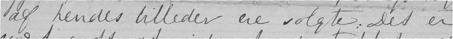af hendes billeder ere solgte. Det er
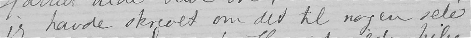jeg havde skrevet om det til nogen selv
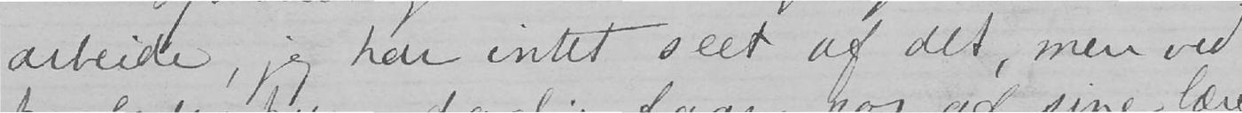arbeide, jeg har intet seet af det, men ved
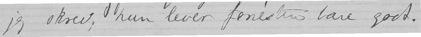jeg skrev, hun lever forresten bare godt.
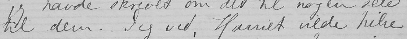til dem. Jeg ved, Harriet vilde hilse
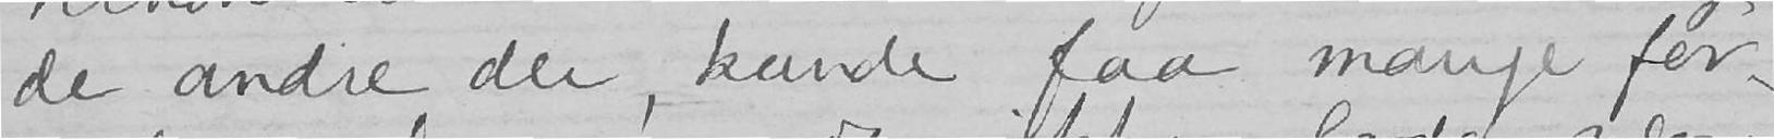de andre der, kunde faa mange for-
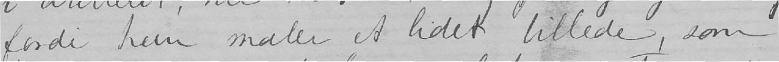fordi hun maler et lidet billede, som
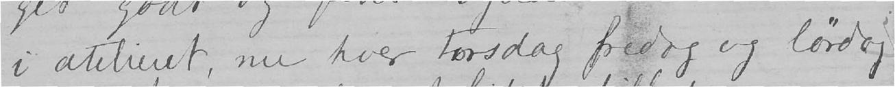i atelieret, nu hver torsdag fredag og lørdag
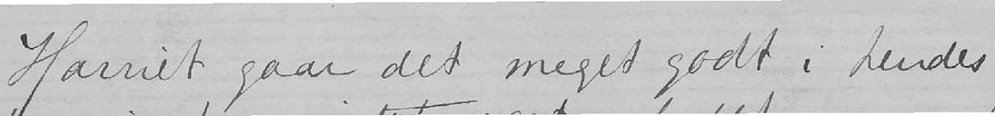Harriet gaar det meget godt i hendes
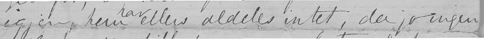igjen, hun har ellers aldeles intet, da jo ingen
